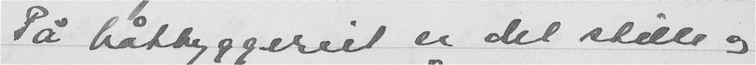På båtbyggeriet er det stille og
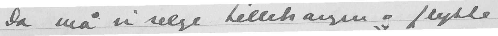Da må vi selge Lillehaugen og flytte
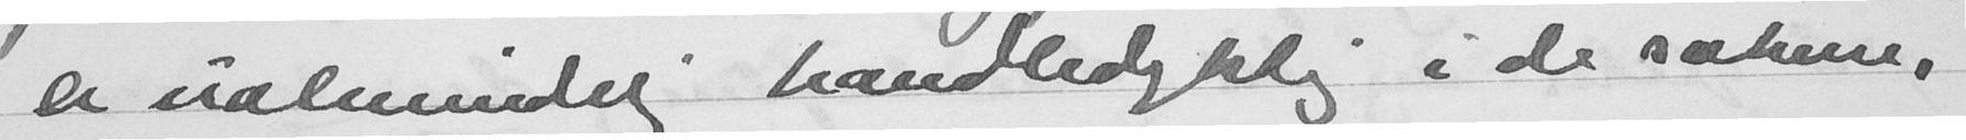er ualmindelig handledyktig i de sakene,
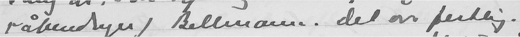råbendryer) Bellmane. Det var festlig.
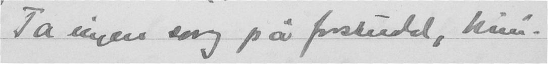Ta ingen sorg på forskudd, Nini-
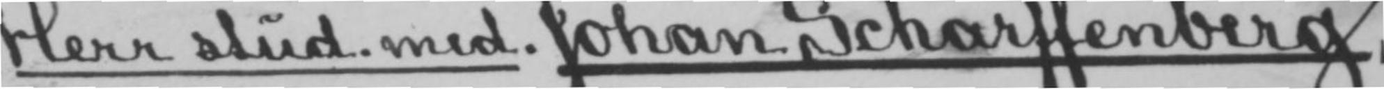Herr stud. med. Johan Scharffenberg,
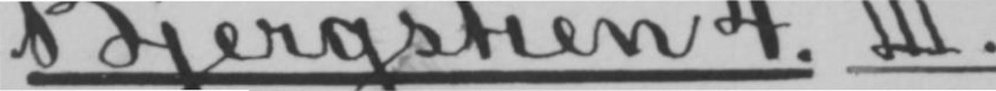Bjergstien 4. III.
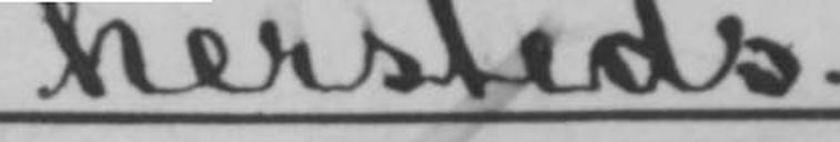hersteds.
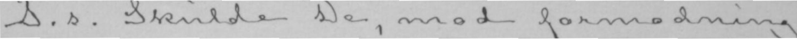P. s. Skulde De, mod formodning,
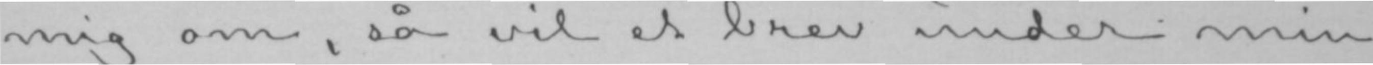mig om, så vil et brev under min
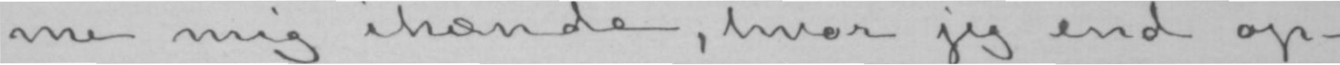me mig ihænde, hvor jeg end op-
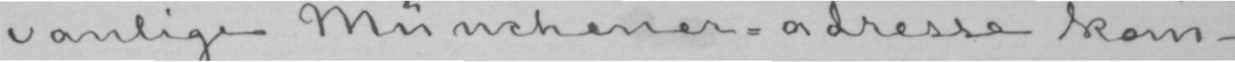vanlige Münchener-adresse kom-
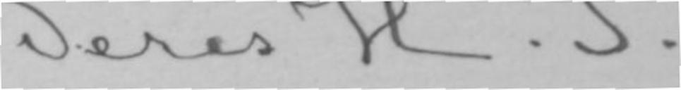Deres H. I.
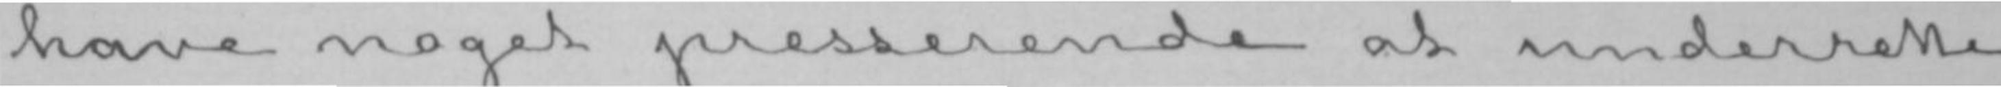have noget presserende at underrette
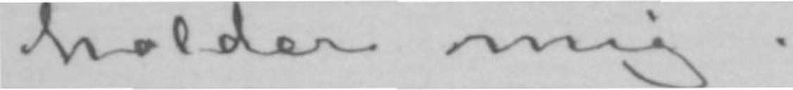holder mig.
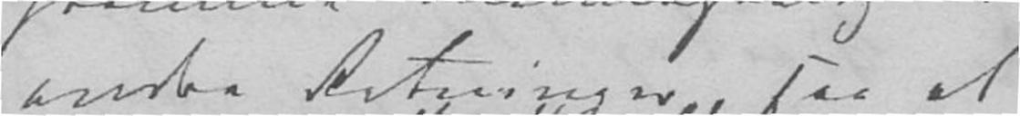andre Retninger, saa at
